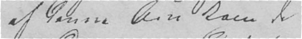af denne Arv kom de
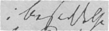i Besiddelse
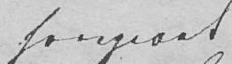foregaaet
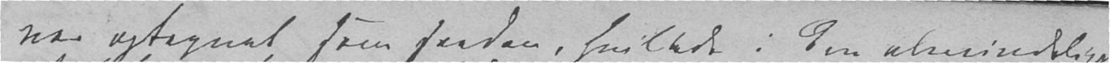var optegnet som saadan, hvilede i den almindelige
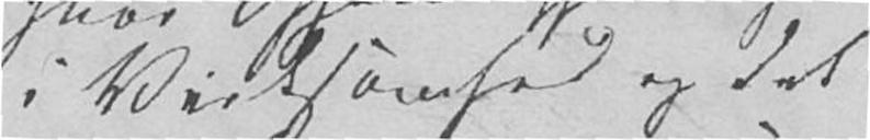i Virksomhed og det
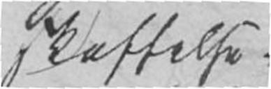skaffelse,
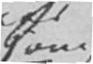som
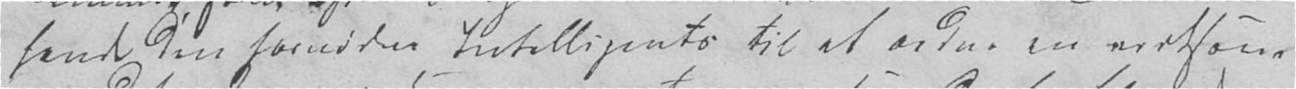havde den fornødne Intelligents til at ordne en virksom
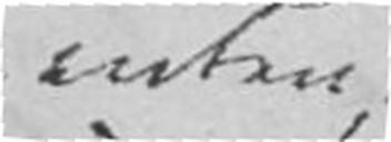enten
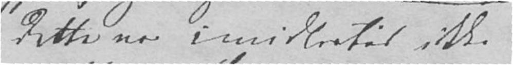Dette var imidlertid ikke
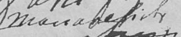Monarchiets
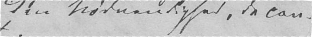den Nødvendighed, de con-
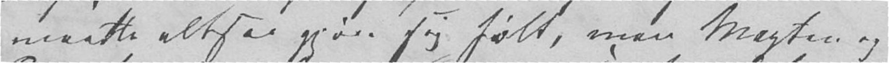maatte altsaa gjøre sig følt, men Magten og
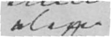alene
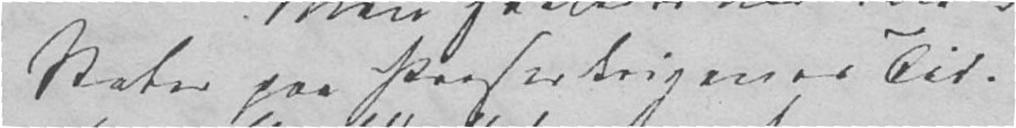Stater paa Perserkrigenes Tid.
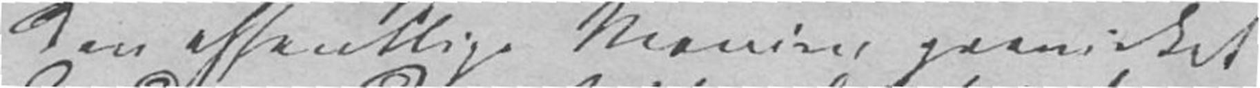den offentlige Mening paavirket
